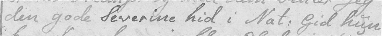den gode Severine hid i Nat. Gid hun
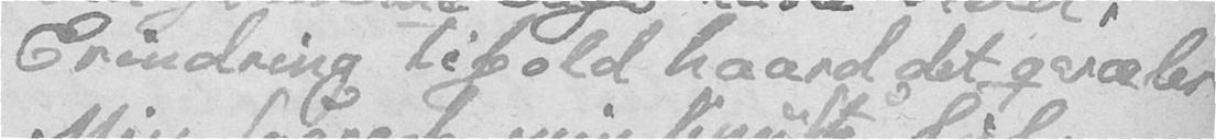Erindring tifold haard det qvæler
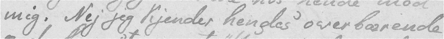mig. Nej jeg kjender hendes overbærende
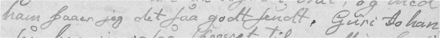ham faaer jeg det saa godt sendt. Guri Johan
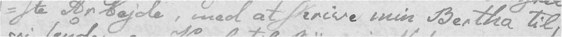-ste Arbejde, med at skrive min Bertha til,
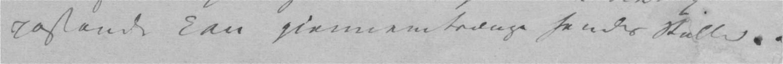passende kan gjennemtrænge hendes Roller.
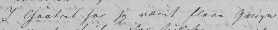I Theatret har jeg været flere Gange
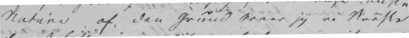Naturer, af den Grund troer jeg vi Norske
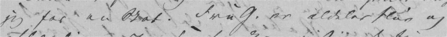jeg for en Skat. Fru G. er aldeles sløv og
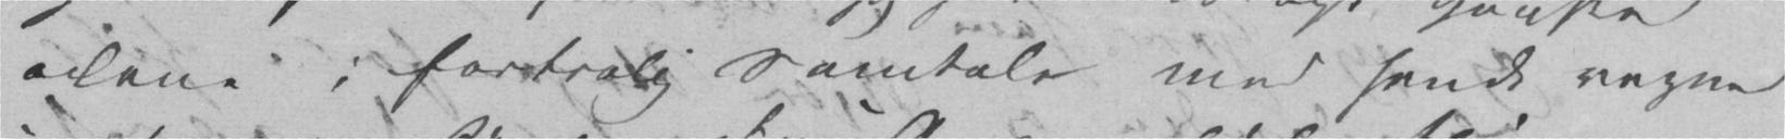alene i fortrolig Samtale med hende regner
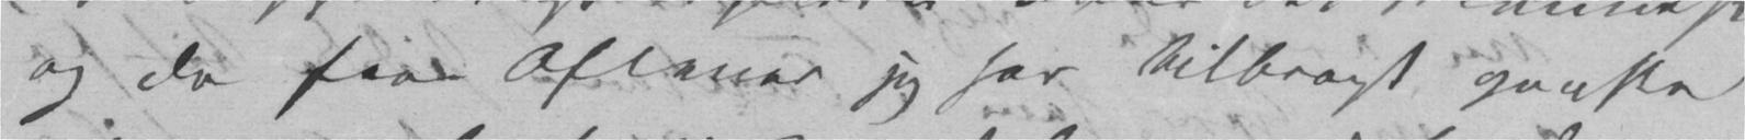og de fire Aftener jeg har tilbragt ganske
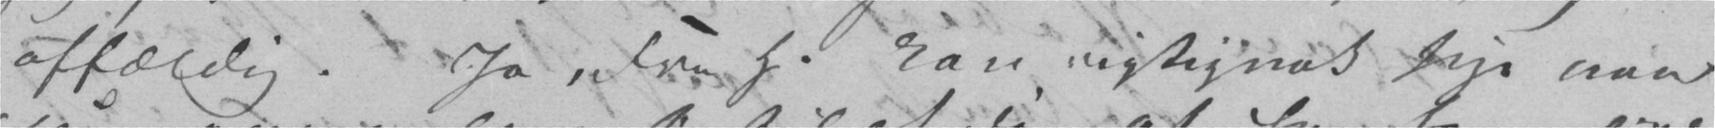affældig. Ja, Fru H. kan rigtignok sige naar
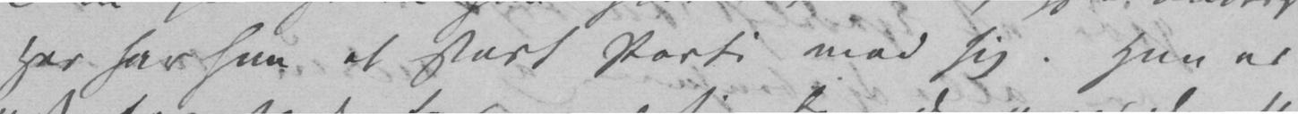Her har hun et stort Parti mod sig. Hun er
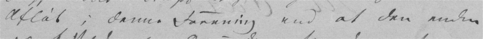Afløb i denne Forening end at den endnu
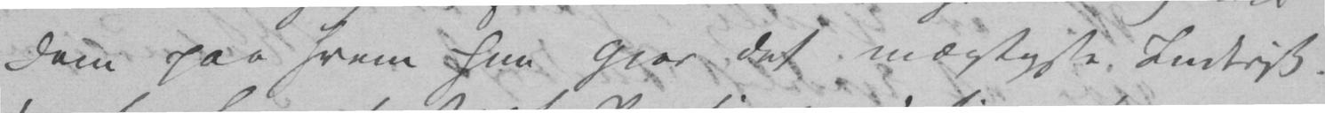dem paa hvem hun gjør det mægtigste Indtryk.
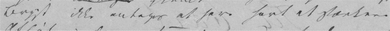Bryst ikke antages at have havt et stærkere
